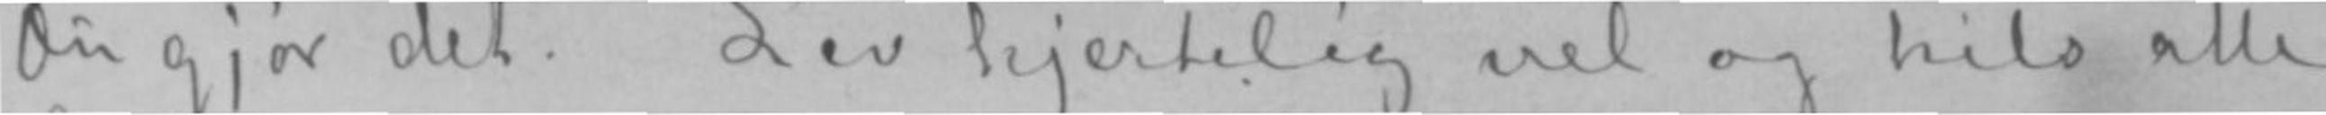Du gjør det. Lev hjertelig vel og hils alle
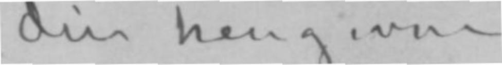din hengivne
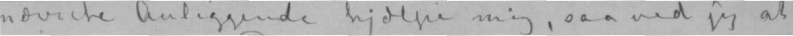nævnte Anliggende hjælpe mig, saa ved jeg at
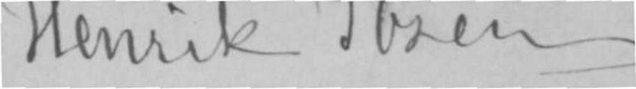Henrik Ibsen.
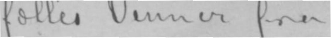fælles Venner fra
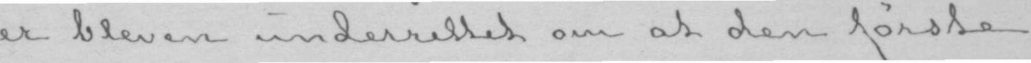er bleven underrettet om at den første
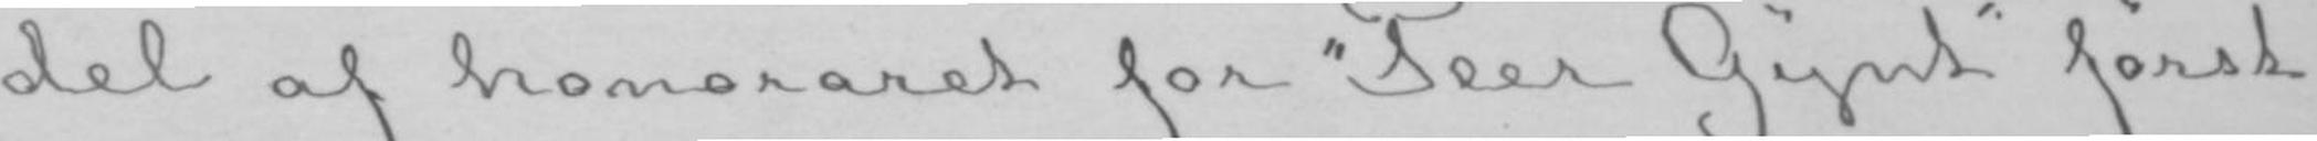del af honoraret for «Peer Gynt» først
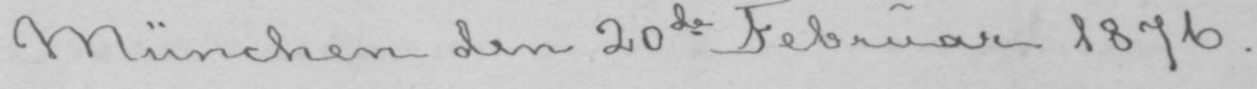München den 20de Februar 1876.
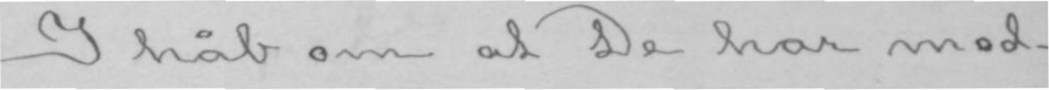I håb om at De har mod-
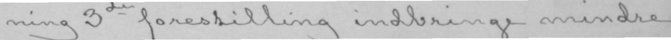ning 3die forestilling indbringe mindre
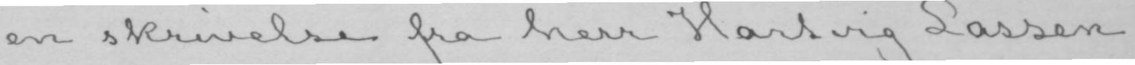en skrivelse fra herr Hartvig Lassen
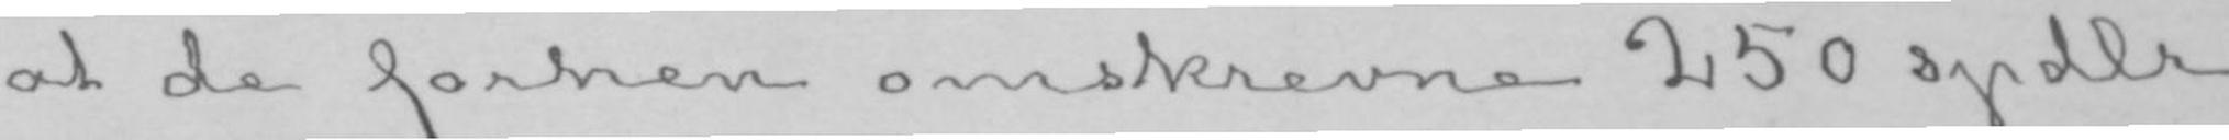at de forhen omskrevne 250 spdlr
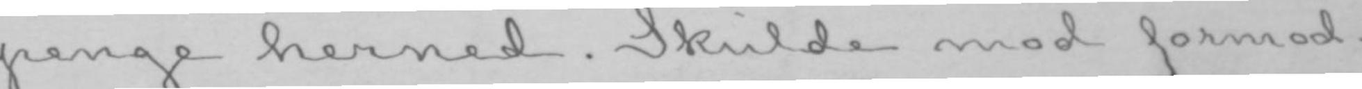penge herned. Skulde mod formod-
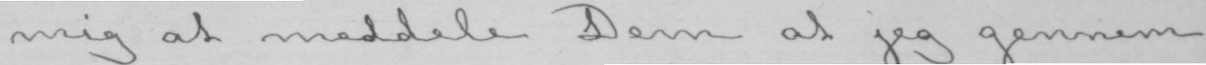mig at meddele Dem at jeg gennem
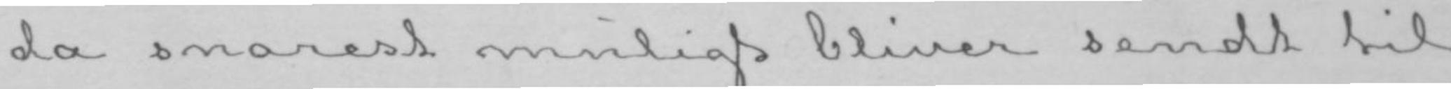da snarest muligt bliver sendt til
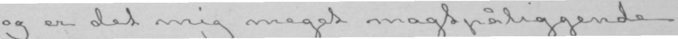og er det mig meget magtpåliggende
